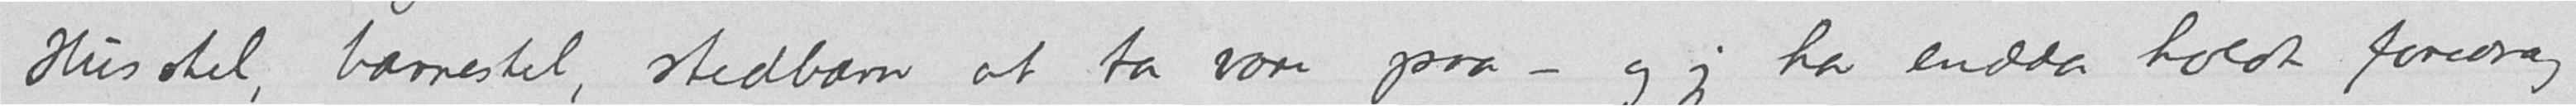Husstel, barnestel, spedbarn at ta vare paa - og jeg har endda holdt foredrag
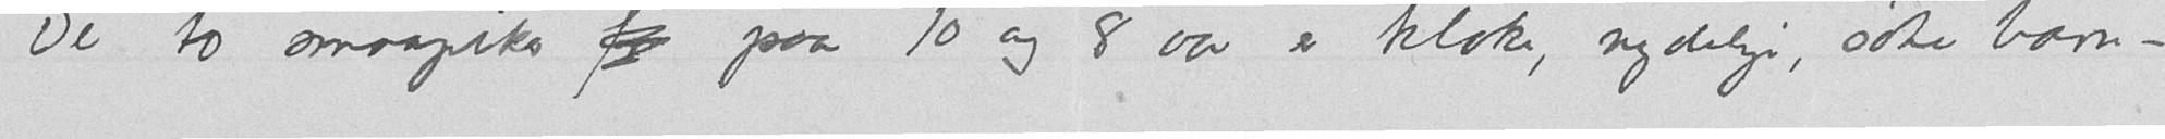De to smaapiker  paa 10 og 8 aar er kloke, nydelige, søde barn -
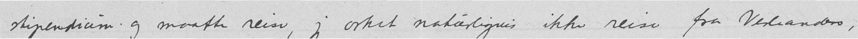stipendium og maatte reise, jeg orket naturligvis ikke reise fra Vesleanders,
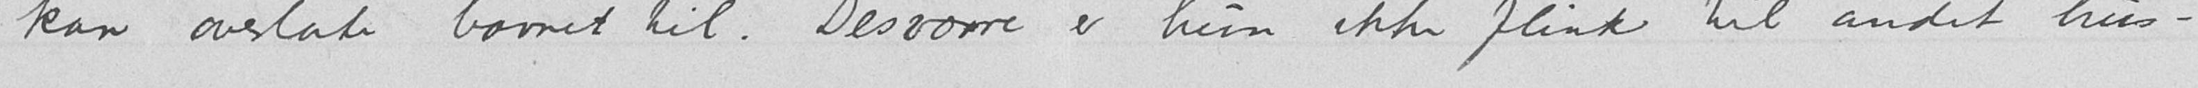kan overlate barnet til. Desværre er hun ikke flink til andet hus-
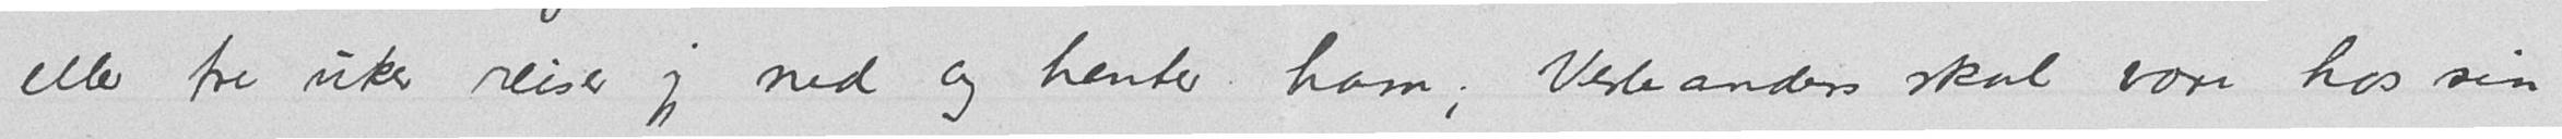eller tre uke reise jeg ned og henter ham; Vesleanders skal være hos sin
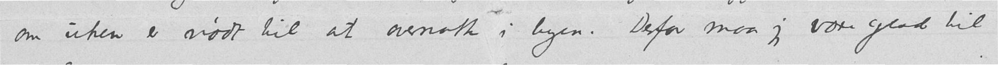om uken er nødt til at overnatte i byen. Defor maa jeg være glad til
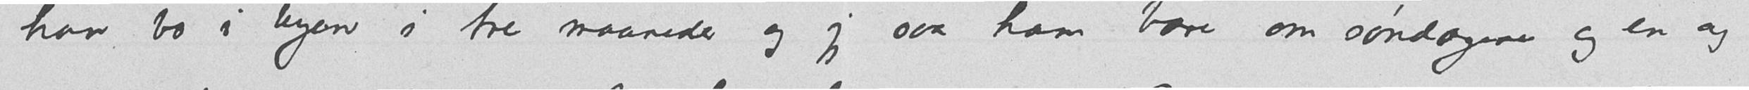han bo i byen i tre maaneder og jeg saa han bare om søndagene og en og
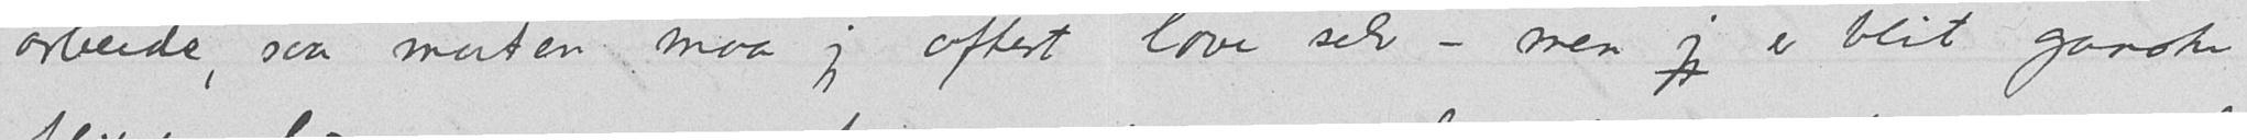arbeide, saa maten maa jeg ofte lage selv - men jeg er blit ganske
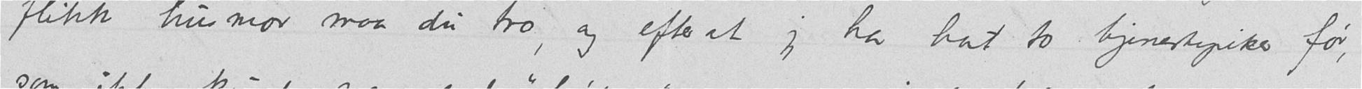flink husmor maa du tro, og efter at jeg har hat to tjenestepiker før,
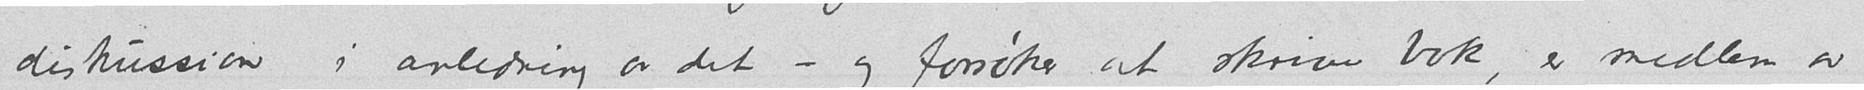diskussion i anledning av det - og forsøker at skrive bok, er medlem av
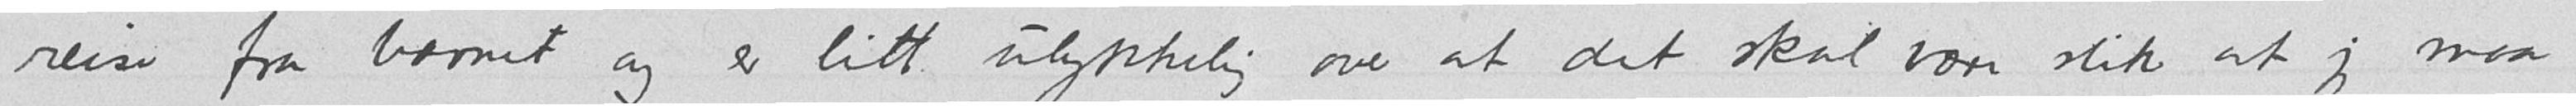reise fra barnet og er litt ulykkelig over at det skal være slik at jeg maa
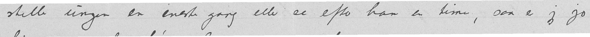stelle ungen er eneste gang eller si efter ham en time, saa er jeg jo
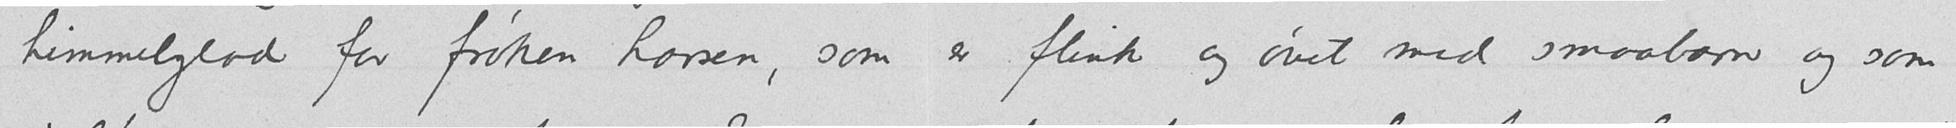himmelglad for frøken Larsen, som er flink og øvet med smaabarn og som
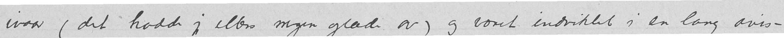ivaar (det hadde jeg ellers megen glæde av) og været indviklet i en lang avis-
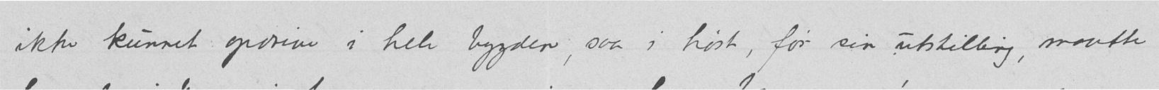ikke kunnet opdrive i hele bygden, saa i høst, før sin utstilling, maatte
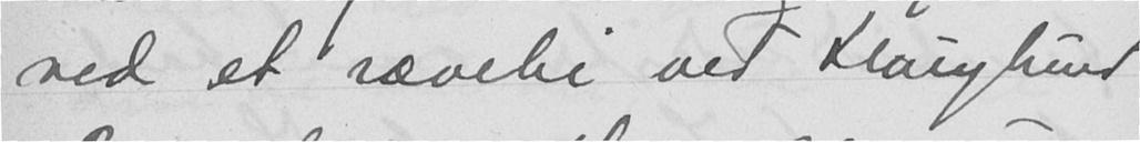ved et rævehi ved Hauglund
